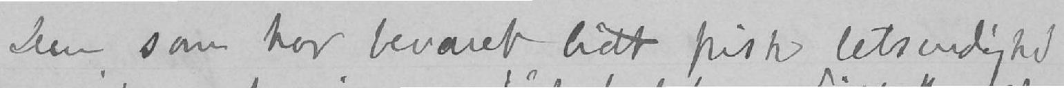Den som har bevaret lidt frisk letsindighed
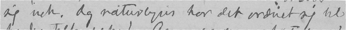sig nok. Og naturligvis har det ordnet sig til
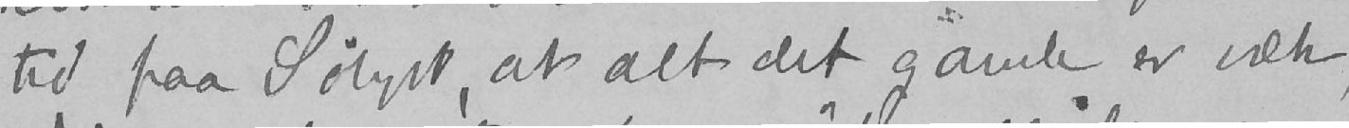tid paa Sølyst, at alt det gamle er væk,
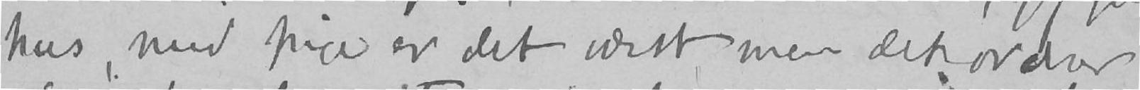hus, med pige er det værst, men det ordner
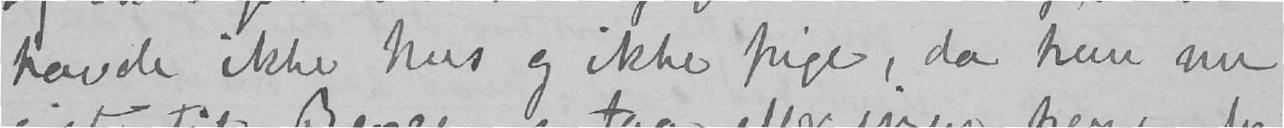havde ikke hus og ikke pige, da hun nu
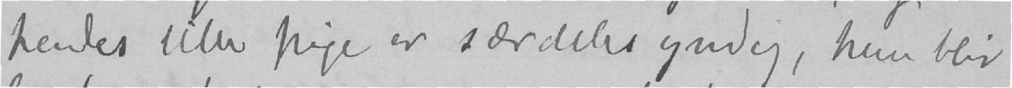hendes lille pige er særdeles yndig, hun blir
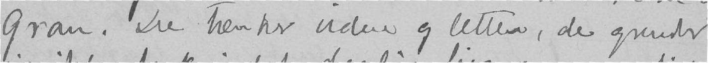Gran. De tænker videre og lerrere, de grunder
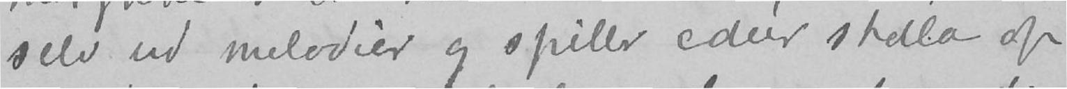selv ud melodier og spiller eders skala op
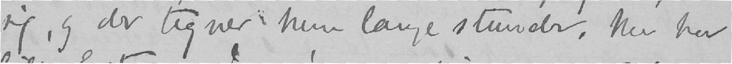sig, og der tegner hun lange stunder. Nu har
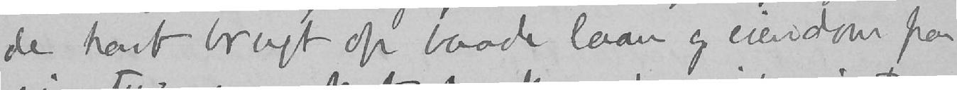de havt brugt op baade laan og eiendom paa
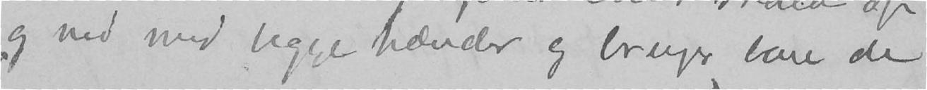og ned med begge hænder og bruger bare de
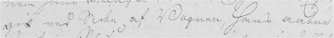gik ved Siden af Vognen, hans aabne
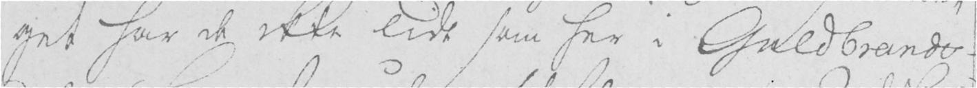get har de ikke lidt som her i Guldbrands-
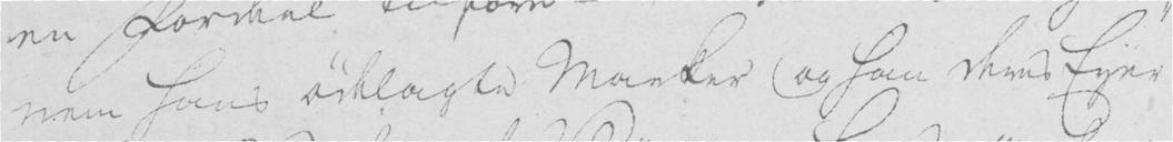nem hans ødelagte Marker og som deres Eyer
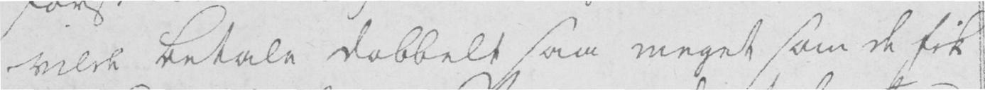vilde betale dobbelt saa meget som de fik
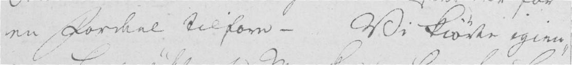en Fordeel tilforn - Vi kiørte igien-
